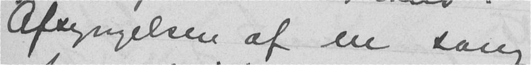Afsyngelsen af en sang
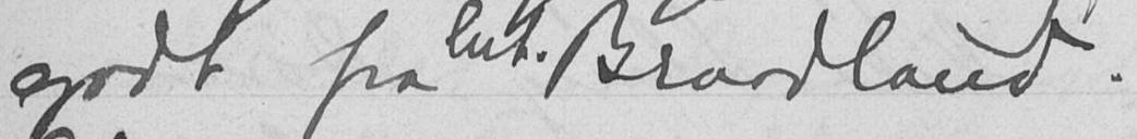godt fra lnt. Braadland.
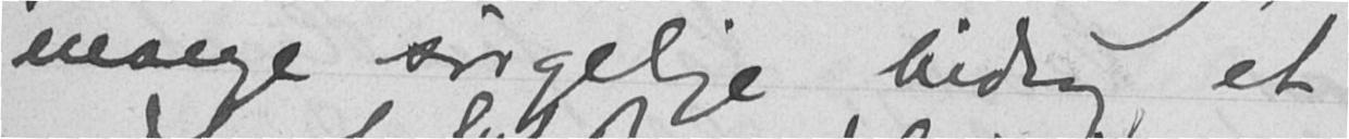mange sørgelige bidrag, et
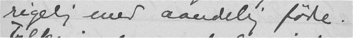rigelig med aandelig føde.
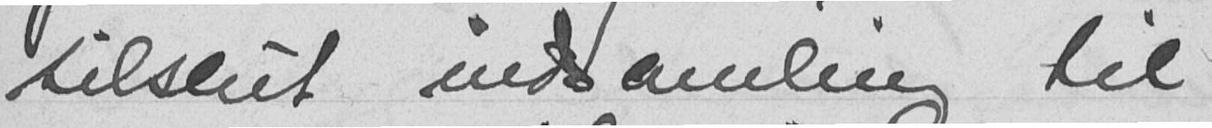tilslut indsamling til
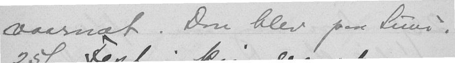vaarnat. Don blev paa Sund.
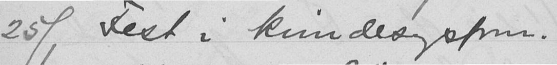25/1 Fest i kvindesagsforn.
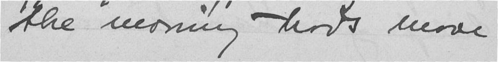the morning trods mave
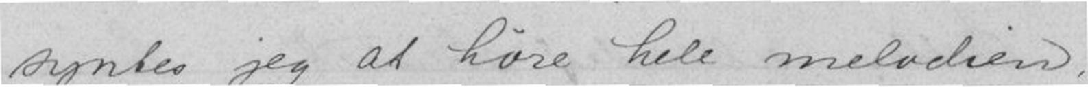syntes jeg at høre hele melodien,
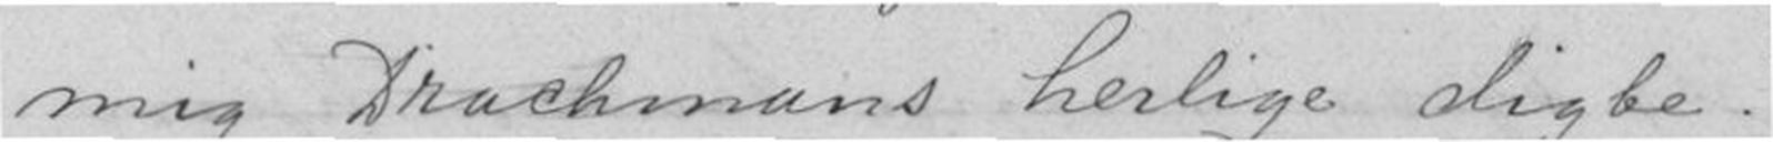mig Drachmans herlige digte!
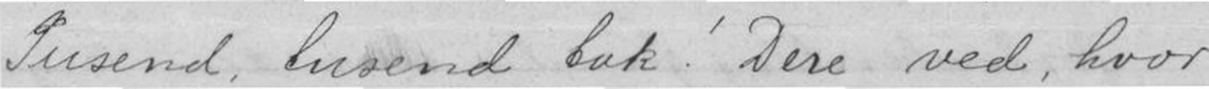Tusend, tusend tak! Dere ved, hvor
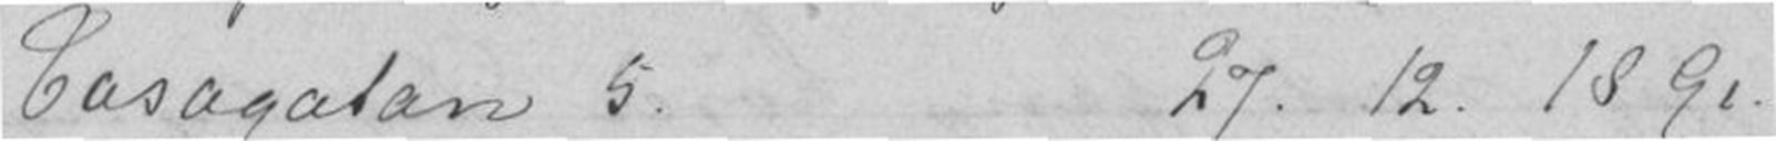Vasagatan 5.27. 12. 1891
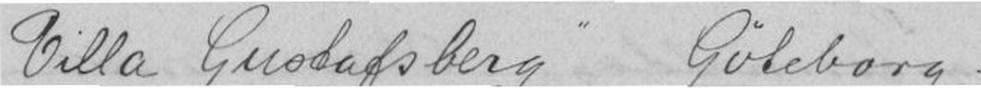"Villa Gustafsberg"Gøteborg
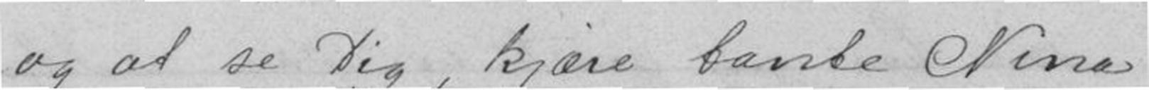og at se Dig, kjære tante Nina
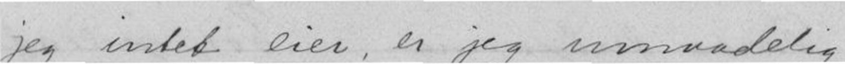jeg intet eier, er jeg umaadelig
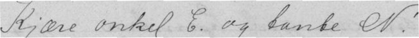Kjære onkel E. og tante N!
